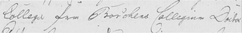Collega fra Borchens Collegium Doctor
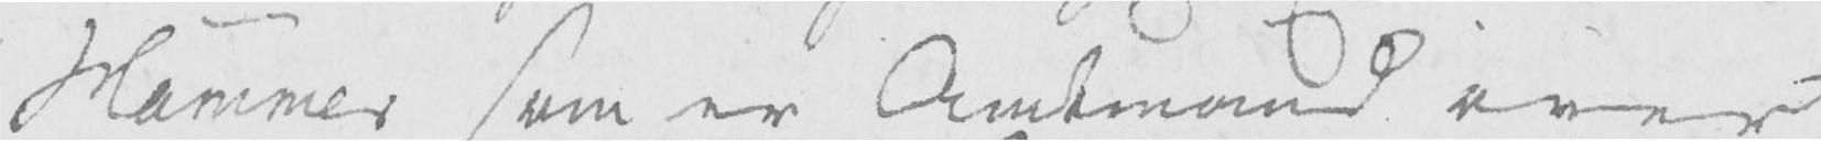Hammer som er Amtmand over
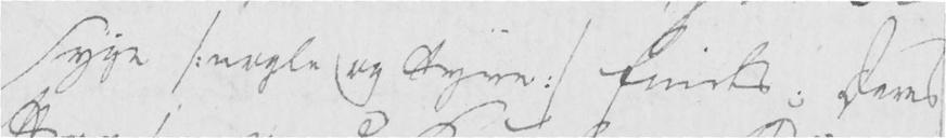syge /: nogle og Tyve :/ findes; Deres
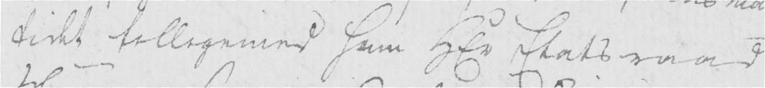tidet tilligemed ham Hr Etatsraad
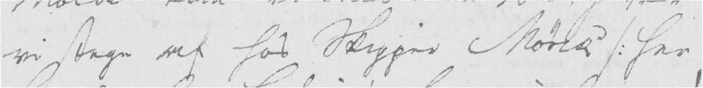vi tage af hos Skipper Mørck /: her
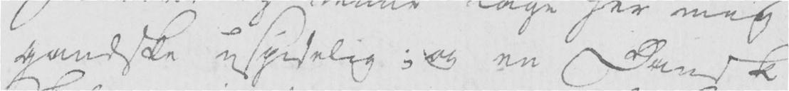gandske uspiselig; og en Dansk
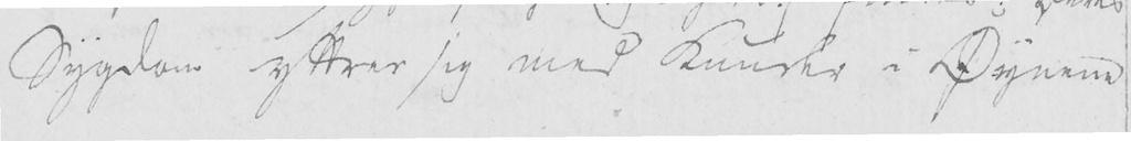Sygdom yttrer sig med kunde i Øynene
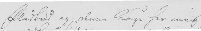Fladbrød og denne Kage her var
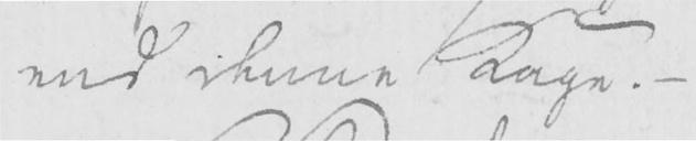end denne Kage. -
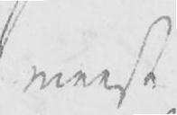meest
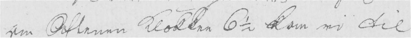om Aftenen Klokken 6 1/2 kom vi til
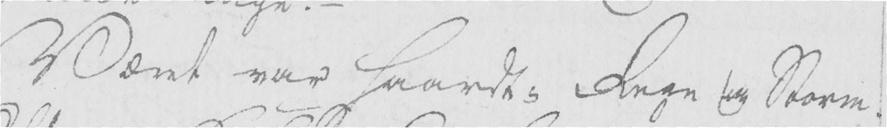Været var haardt; Regn og Storm.
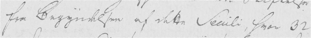fra begyndelsen af dette Seculi, hvor 32
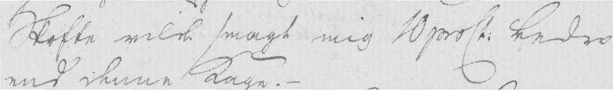Skafte vilde smagt mig fl prost. bedre
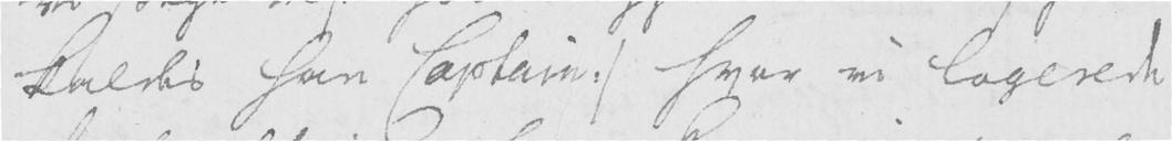kaldes han Captain :/ hvor vi logerede
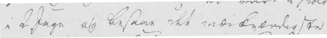i 2 dage og besaae det mærkværdigste
